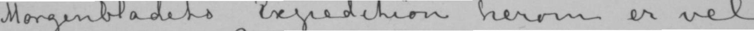Morgenbladets Expedition herom er vel
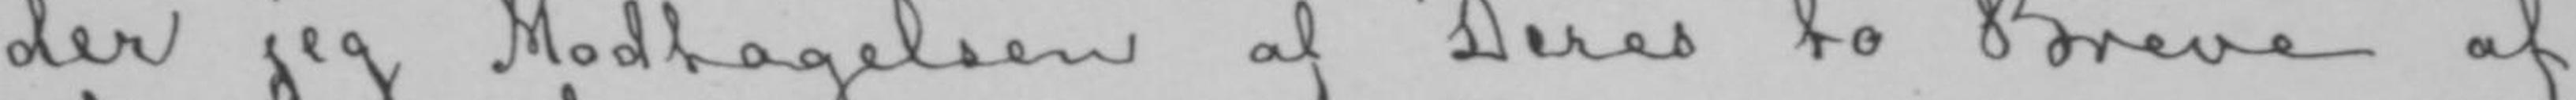der jeg Modtagelsen af Deres to Breve af
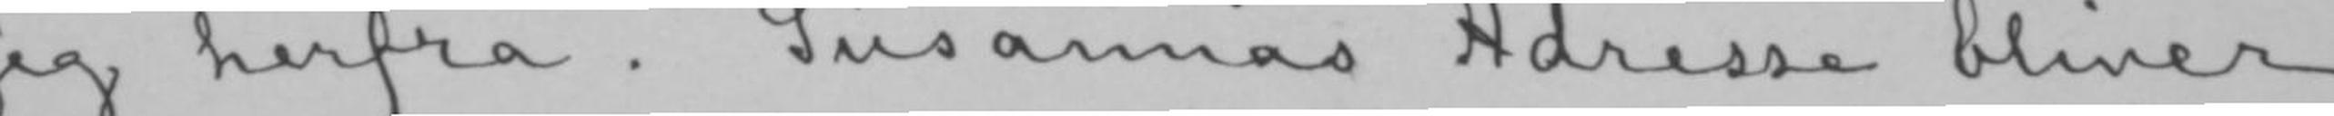jeg herfra. Susannas Adresse bliver
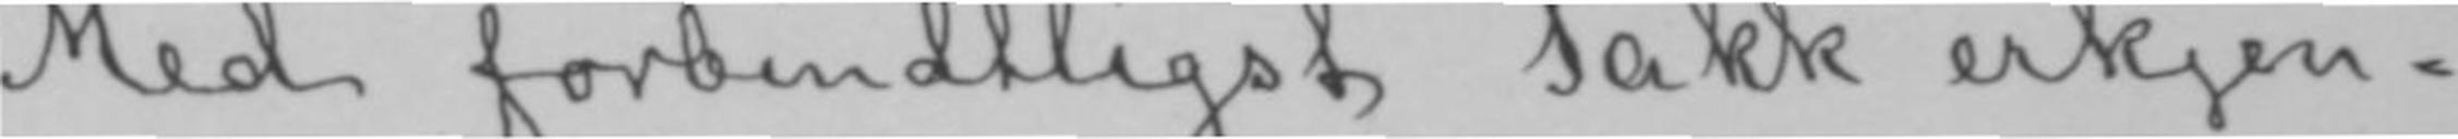Med forbindtligst Takk erkjen-
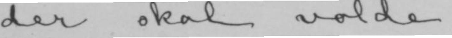der skal volde.
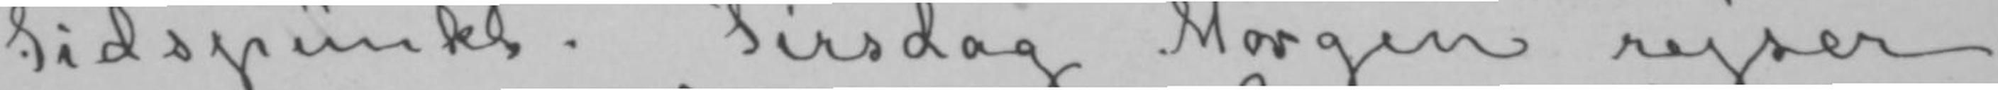Tidspunkt. Tirsdag Morgen rejser
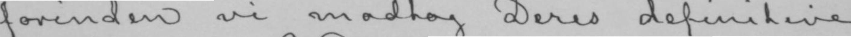forinden vi modtog Deres definitive
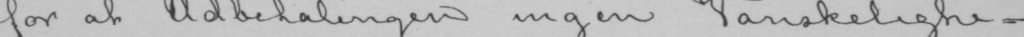for at Udbetalingen ingen Vanskelighe-
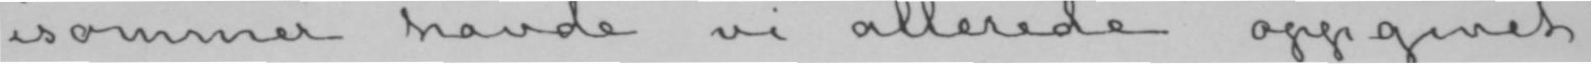isommer havde vi allerede oppgivet
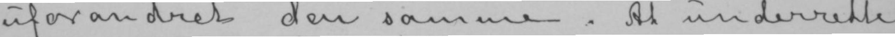uforandret den samme. At underrette
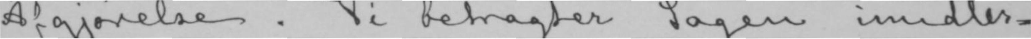Afgjørelse. Vi betragter Sagen imidler-
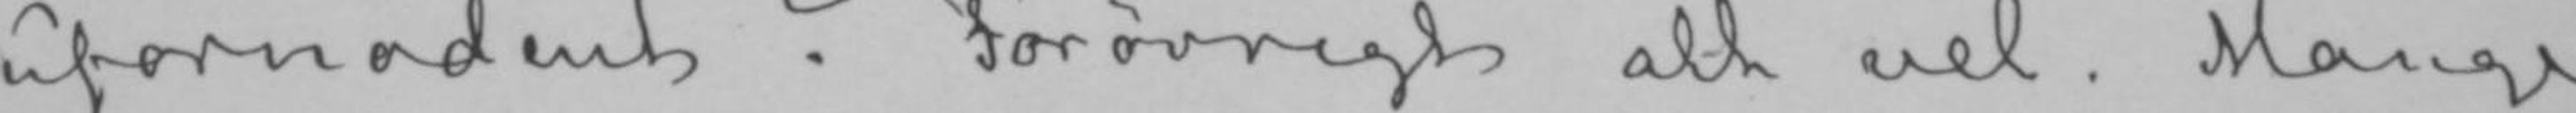ufornødent? Forøvrigt alt vel. Mange
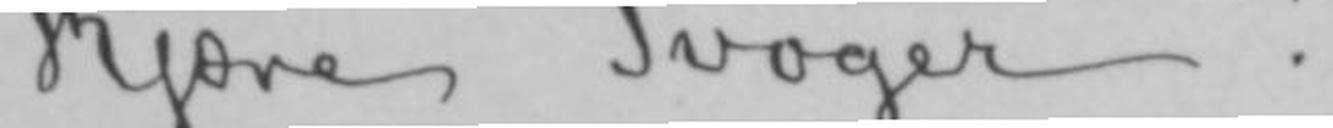Kjære Svoger!
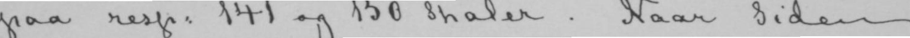paa resp: 141 og 150 Thaler. Naar Tiden
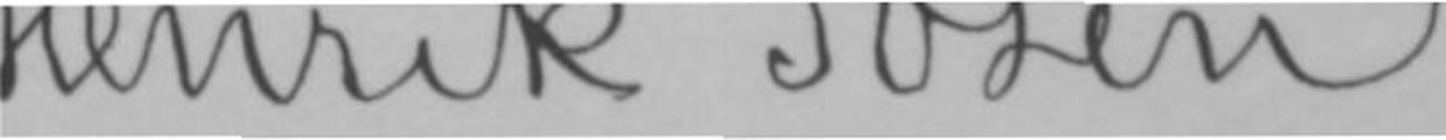Henrik Ibsen.
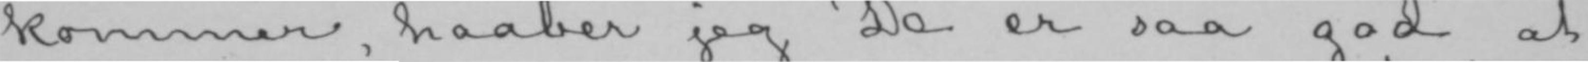kommer, haaber jeg De er saa god at
